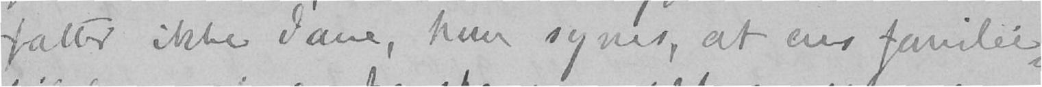fatter ikke Jane, hun synes, at ens familie-
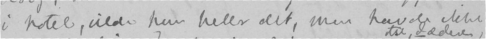i hotel, vilde hun heller det, men havde ikke
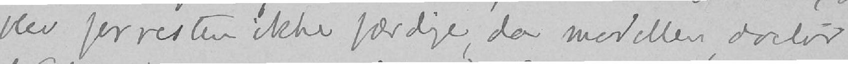blev forresten ikke færdige, da modellen, doctor
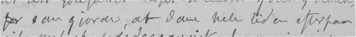for som gjorde, at Jane hele tiden efterpaa
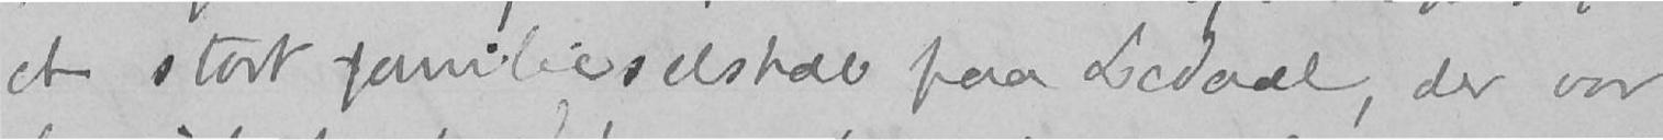et stort familieselskab paa Ledaal, der var
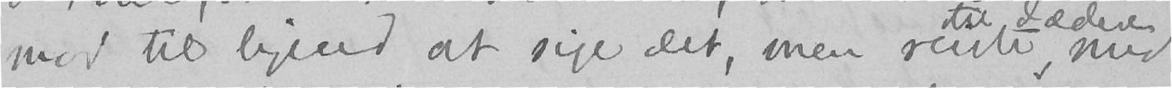mod til ligeend at sige det, men reiste med
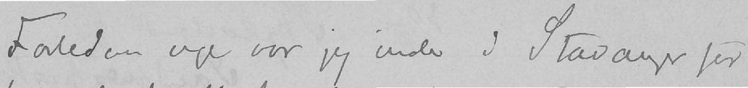Forleden uge var jeg inde i Stavanger for
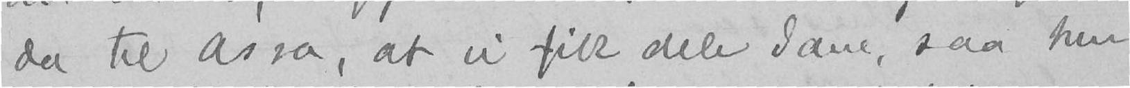da til at Assa, at vi fik dele Jane, saa hun
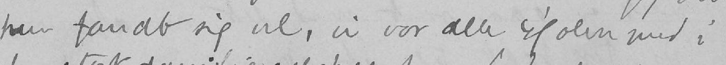hun fandt sig vel, vi var alle Holm med i
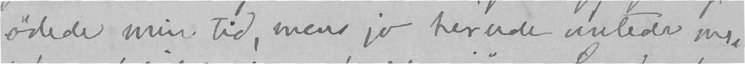ødede min tid, mens jo herude ventet me-
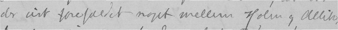der vist forefaldet noget mellem Holm og Allik,
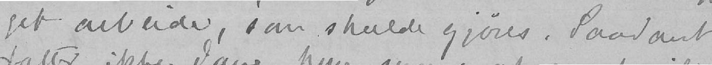get arbeide, som skulde gjøres. Saadant
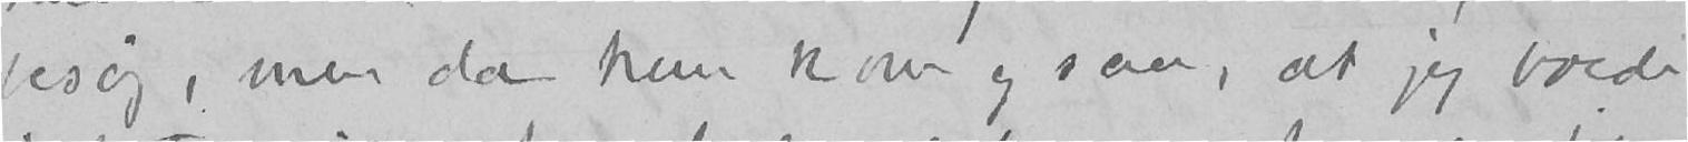besøg, men da hun kom og saa, at jeg boede
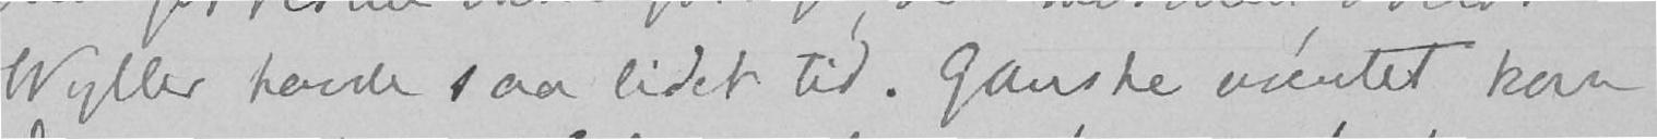Wyller havde saa lidet tid. Ganske uventet kom
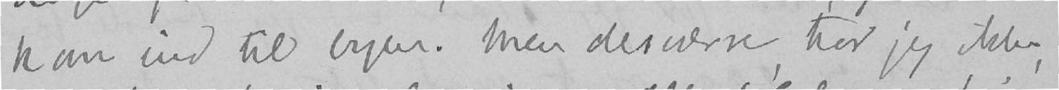kom ind til byen. Men desværre tror jeg ikke
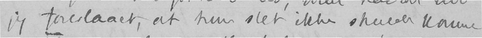jeg foreslaaet, at hun slet ikke skulde komme
